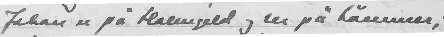Johan er på Holmgild og ser på tømmer,
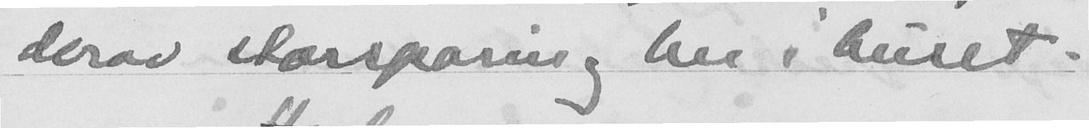derav storsparing her i huset.
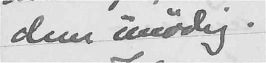dem unødig.
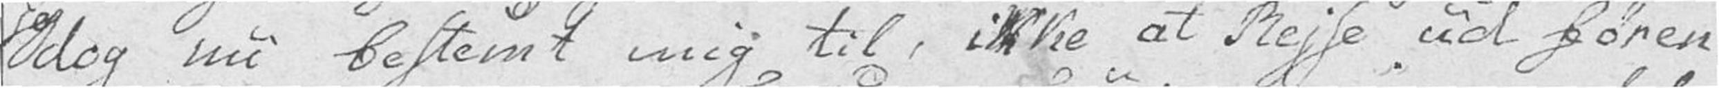jeg dog nu bestemt mig til, ikke at Rejse ud føren
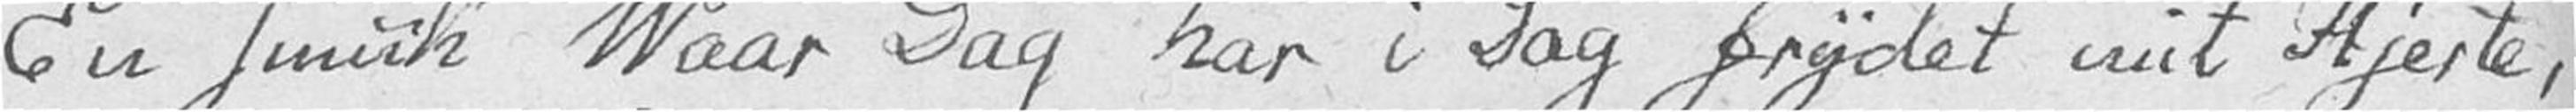En smuk Vaar Dag har i Dag frydet mit Hjerte,
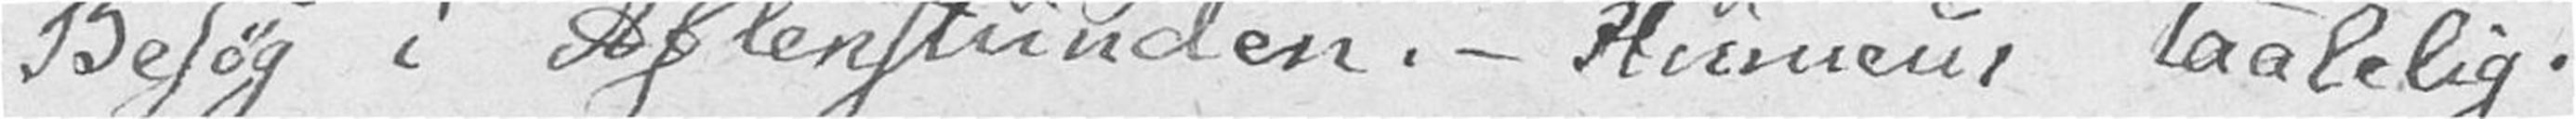Besøg i Aftenstunden. – Humeur taalelig.
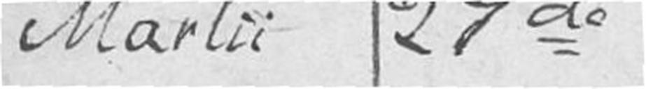Martii 27de
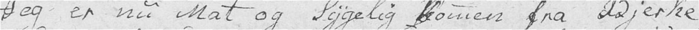Jeg er nu Mat og Sygelig kommen fra Bjerke
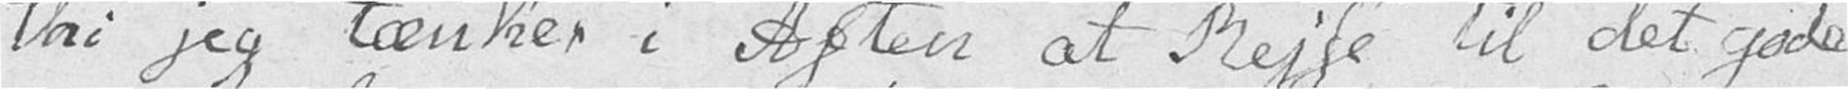thi jeg tænker i Aften at Rejse til det gode
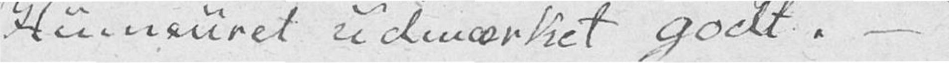Humeuret udmærket godt. –
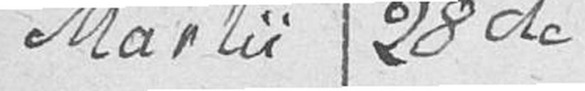Martii 28de
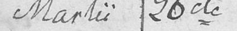Martii 26de
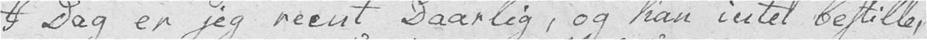I Dag er jeg reent Daarlig, og kan intet bestille,
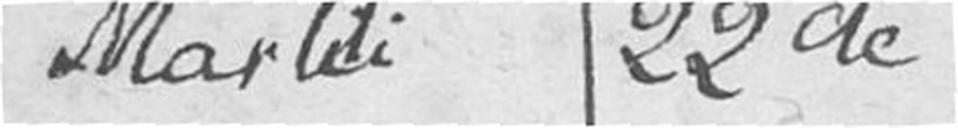Martii 22de
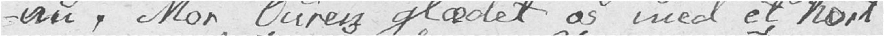-nu, Mor Ouren glædet os med et kort
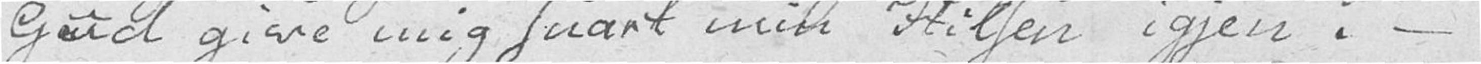Gud give mig snart min Hilsen igjen. –
